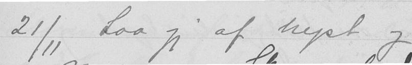21/11 Laa jeg af bryst og
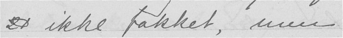21 ikke fakket, men
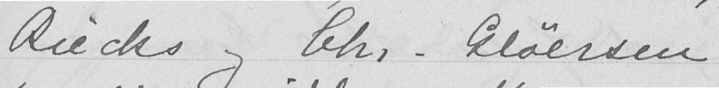Riecks og Chr. Gløersen
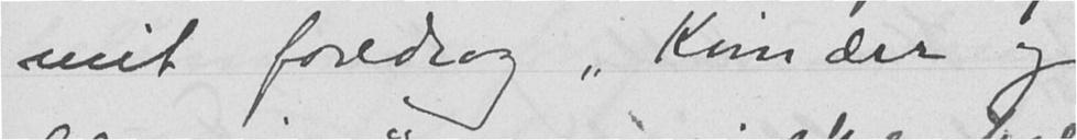mit foredrag "Kvinder og
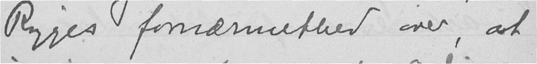Rogges fornærmethed over, at
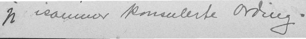jeg isommer konsulerte Ording.
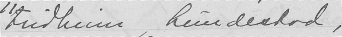Fridheim, Lundestad,
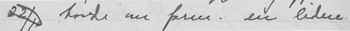22/11 havde om form. en liden
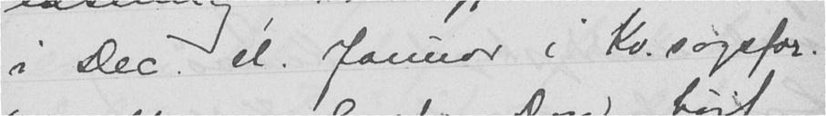i Dec. el. Januar i Kv.sagsfor.
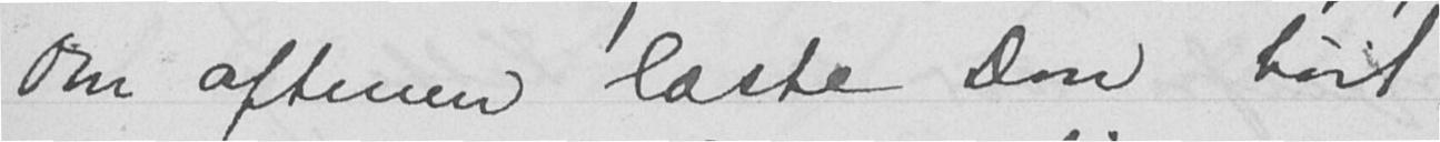Om aftenen læste Don høit
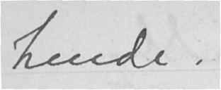hende.
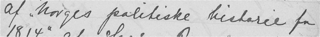af "Norges politiske historie fra
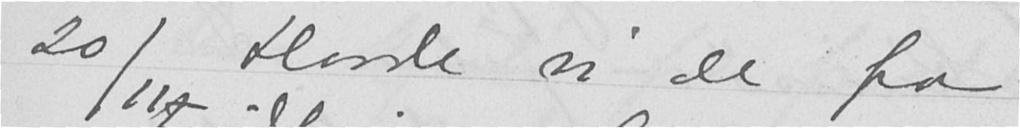20/11 Havde vi de fra
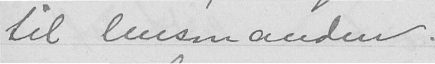til lensmanden.
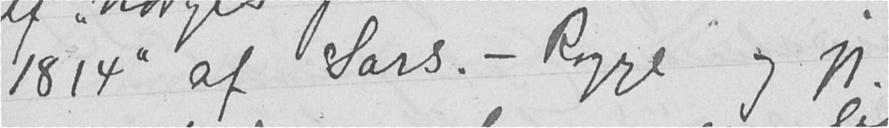1814" af Sars. - Rogge og jeg
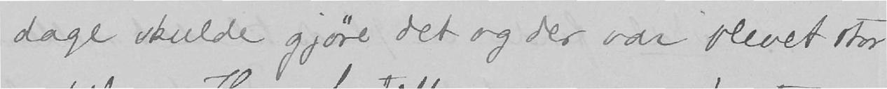dage skulde gjøre det og der var blevet stor
